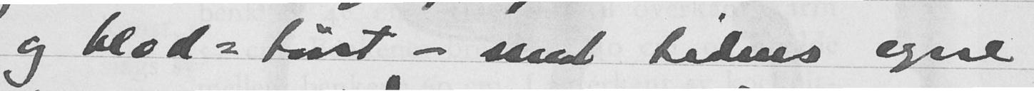og blod-tørst - med tidens egne
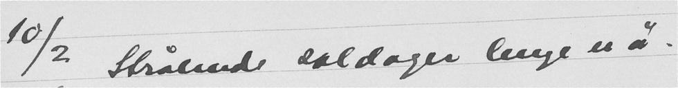10/2 Strålende soldager lenge nå.
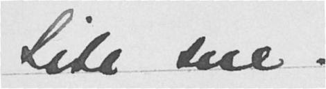Lite sne.
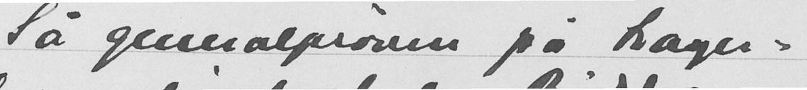Så generalprøven på Lager-
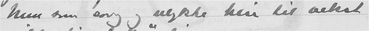Men som sorg og ulykke blir til vekst
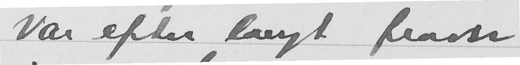Var efter langt fearse
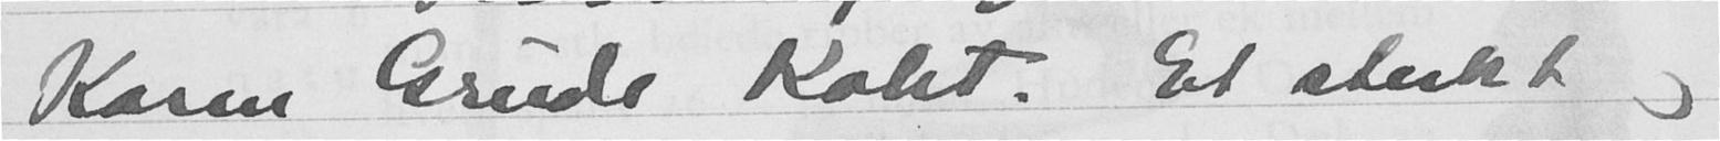Karen Grude Koht. Et sterkt og
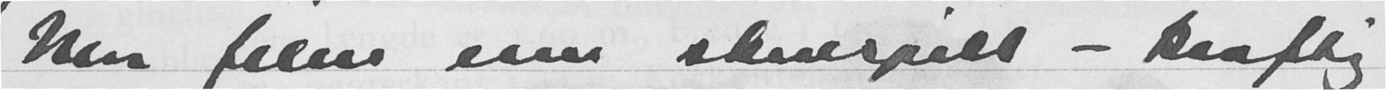Mer film enn skuespill - kraftig
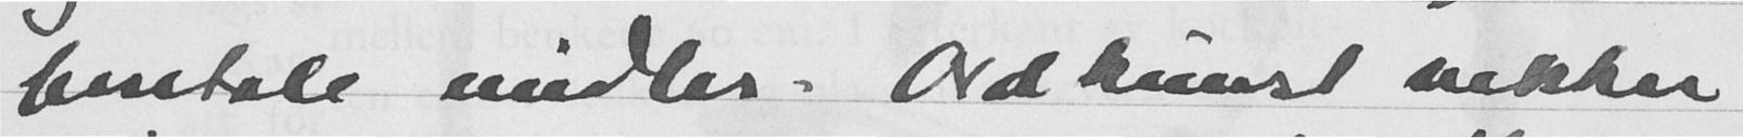brutale midler. Ordkunst vekker
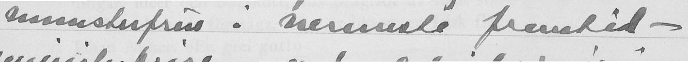minsteren i nermeste fremtid -
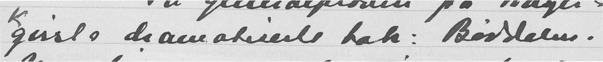qvists dramatiske bok: Bøddelen.
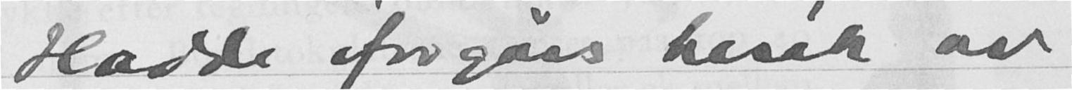Hadde iforgårs besøk av
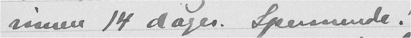innen 14 dager. Spennende!
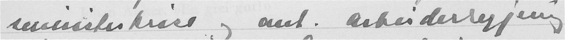senisteskross og ant. arbeiderregjering
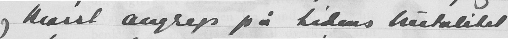og krasst angrep på tidens butalitet
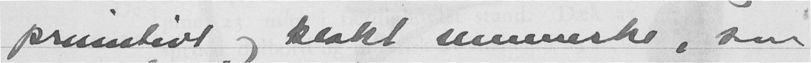primitivt og klokt menneske, som
